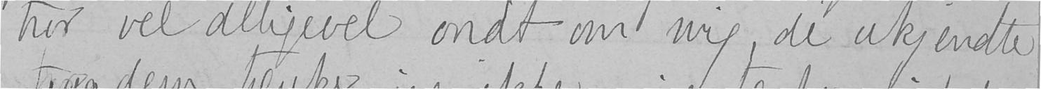tror vel alligevel ondt om mig, de ukjendte
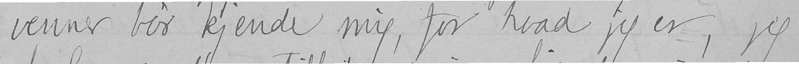venner bør kjende mig, for hvad jeg er, jeg
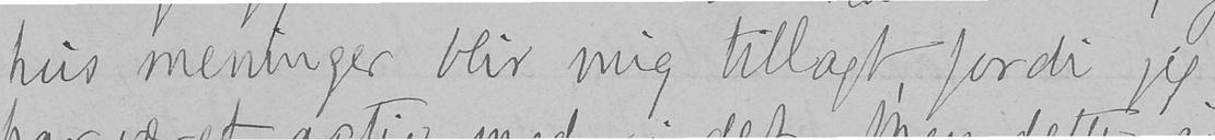hvis meninger blir mig tillagt, fordi jeg
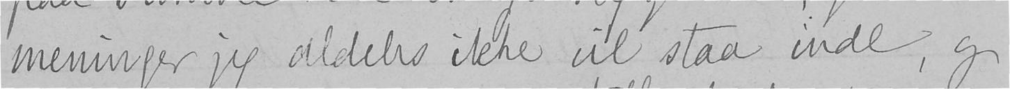meninger jeg aldeles ikke vil staa inde, og
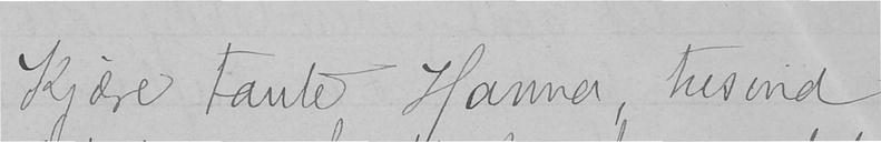Kjære tante Hanna, tusind
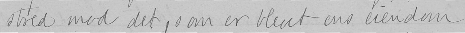stred mod det, som er blevet ens eiendom
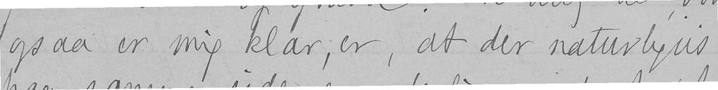ogsaa er mig klar, er, at der naturligvis
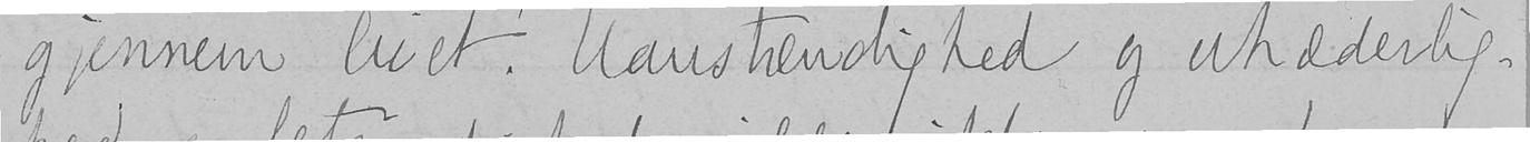gjennem livet. Uanstendighed og uhæderlig-
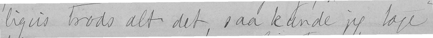ligvis trods alt det, saa kunde jeg tage
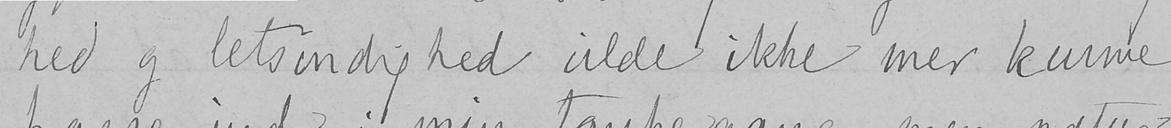hed og letsindighed vilde ikke mer kunne
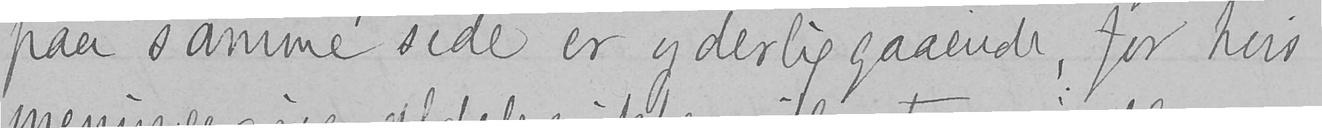paa samme side er yderliggaaende, for hvis
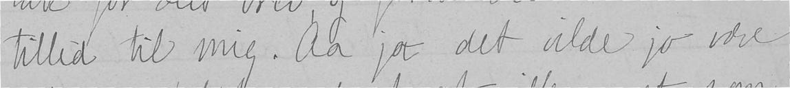tillid til mig. Aa ja det vilde jo være
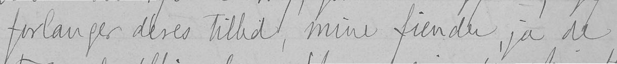forlanger deres tillid, mine fiender, ja de
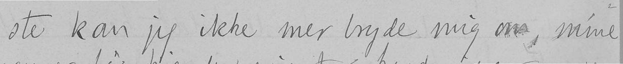ste kan jeg ikke mer bryde mig om, mine
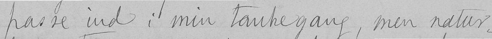passe ind i min tankegang, men natur-
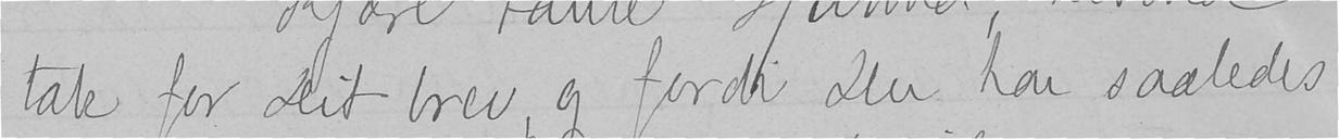tak for Dit brev og fordi Du har saaledes
